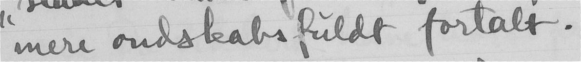mere ondskabsfuldt fortalt.
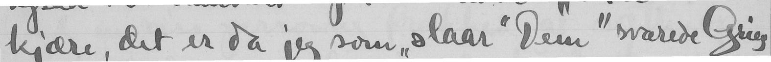kjære, det er da jeg som "slaar" Dem" svarede Grieg
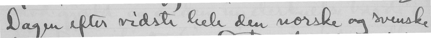Dagen efter vidste hele den norske og svenske
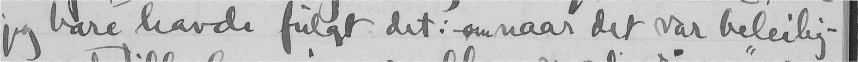jeg bare havde fulgt det: naar det var beleilig-
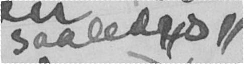saaledes"
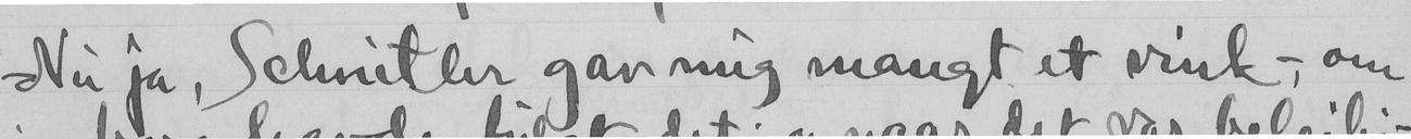Nu ja, Schritler gav mig mangt et vink, om
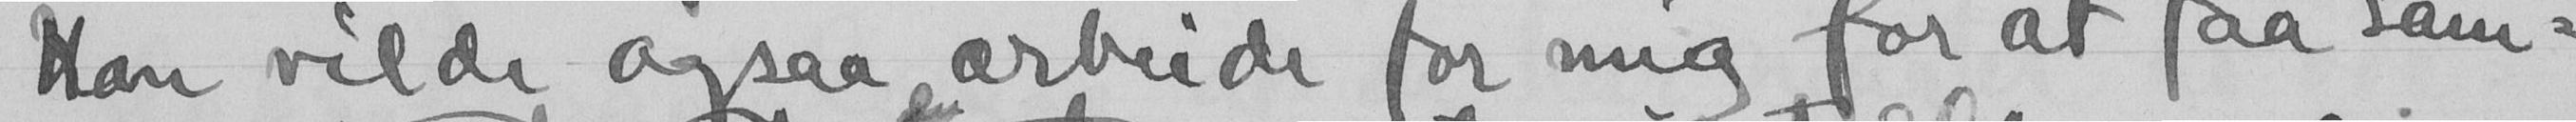Han vilde ogsaa arbeide for mig for at faa sam-
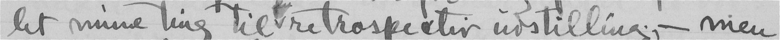let mine ting til retrospectiv udstilling,- men
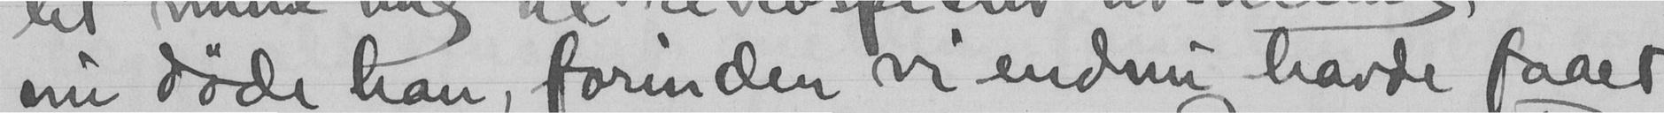nu døde han, forinden vi endnu havde faaet
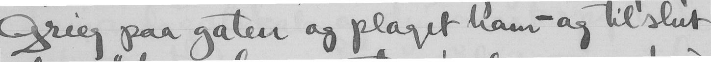Grieg paa gaten og plaget ham - og tilslut
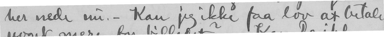her nede nu. - Kan jeg ikke faa lov at betale
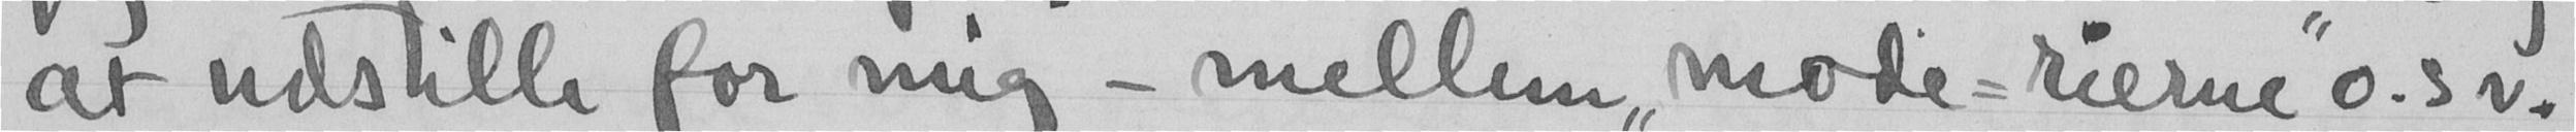at udstille for mig - mellem "mode-rierne" o.s.v.
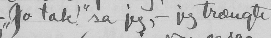- "Jo tak!" sa jeg, - jeg trængte
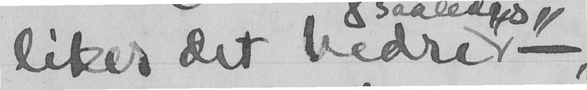liker det bedre, -
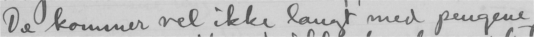De kommer vel ikke langt med pengene
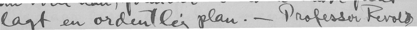lagt en ordentlig plan. - Professor Revold
